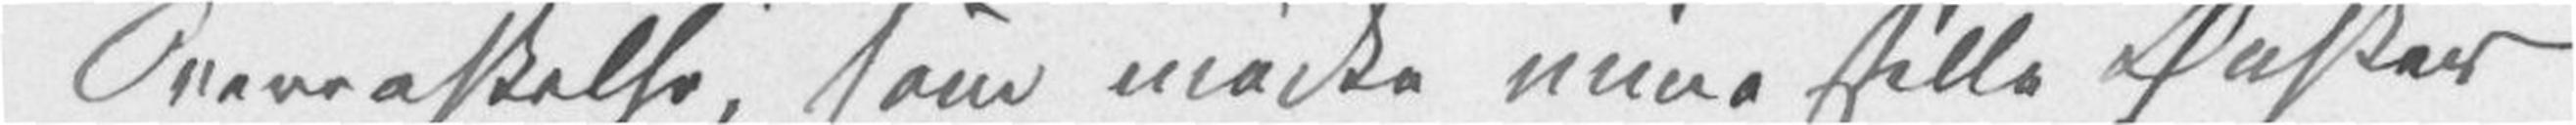Overraskelse, som mødte mine stille Ønsker
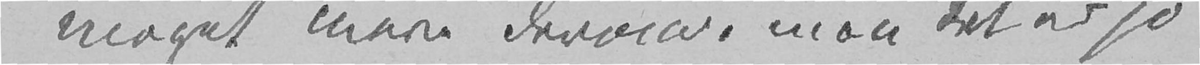meget mere derom, men det er jo
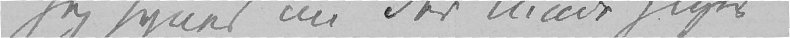Jeg synes nu der kunde siges
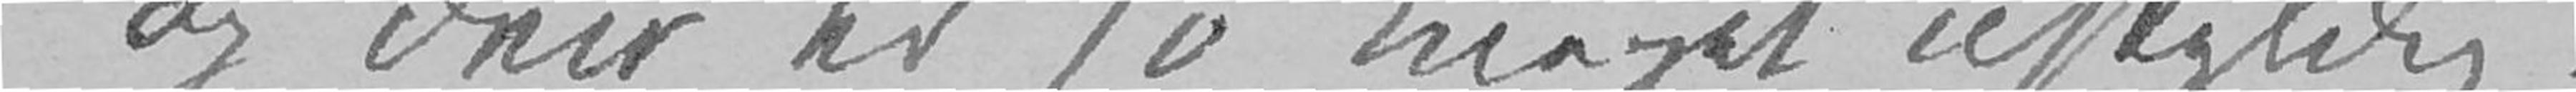og den er jo meget uskyldig.
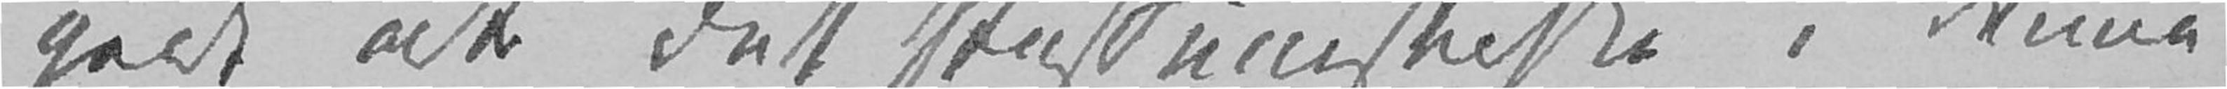godt at «det Pessimistiske» i denne
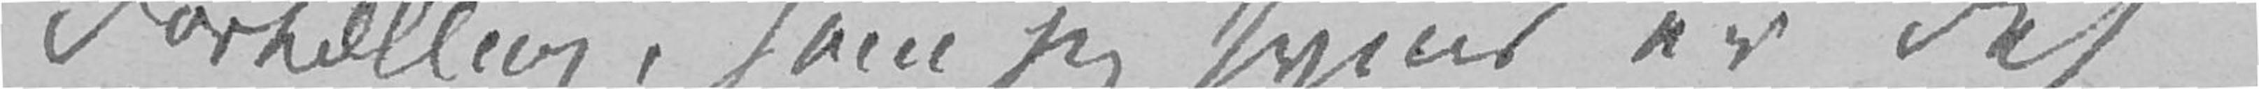Fortælling, som jeg synes er det
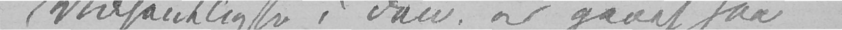Væsentligste i den, er gaaet saa
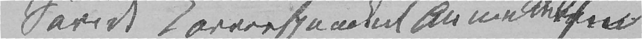Såvidt Korresspondentanmeldelsen
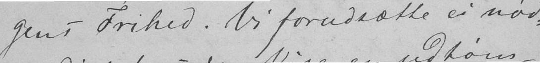gens Frihed. Vi forudsætte ei nød-
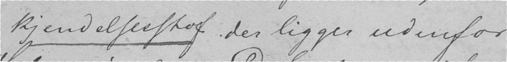kjendelsesstof, der ligger udenfor
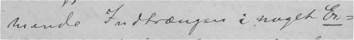mende Indtrængen i noget Er-
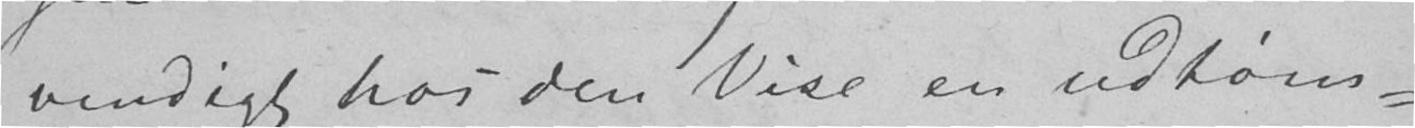vendigt hos den Vise en udtøm-
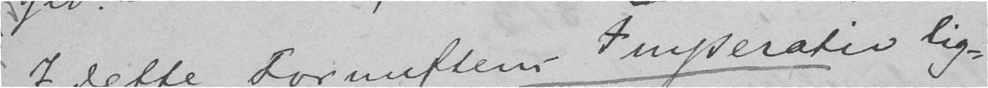I dette Fornuftens Imperativ lig-
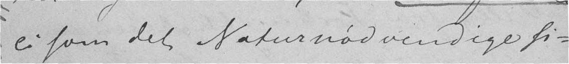ei som det Naturnødvendige si-
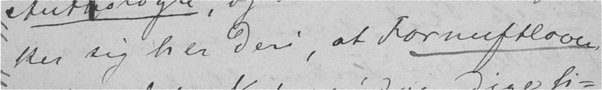ker sig her deri, at Fornuftloven
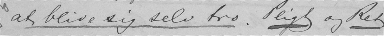at blive sig selv tro. Pligt og Ret
